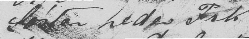Søster heder Frk,
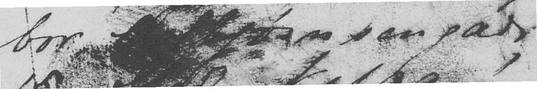bor i Colbjørnsen gade
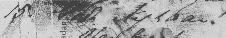15. Godt Nytaar!
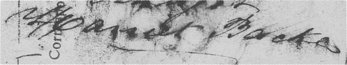Harriet Backer
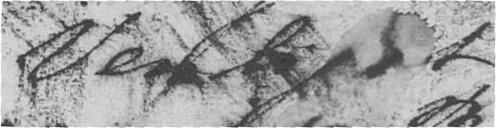Venligst
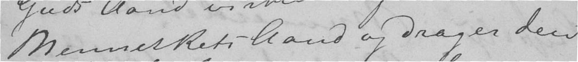Menneskets Aand og drager den
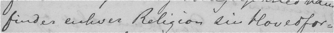finder enhver Religion sin Hovedfor-
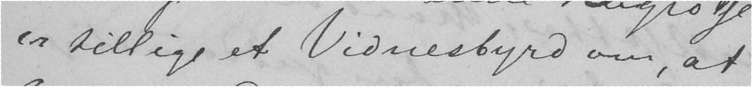er tillige et Vidnesbyrd om, at
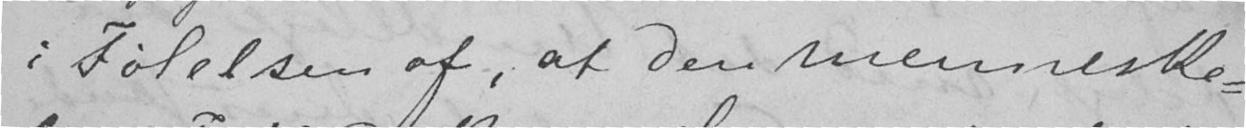i Følelsen af, at den menneske-
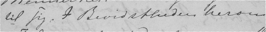til sig. I Bevidstheden herom
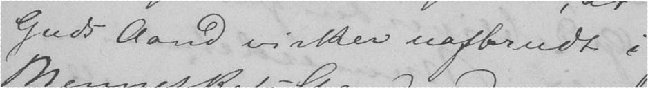Guds Aand virker uafbrudt i
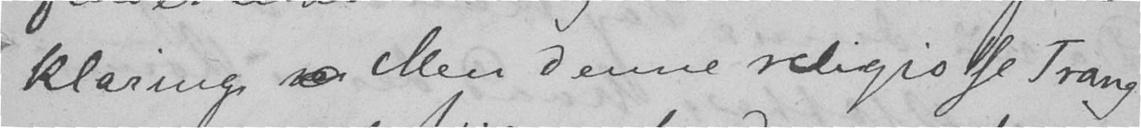klaring. Men denne religiøse Trang
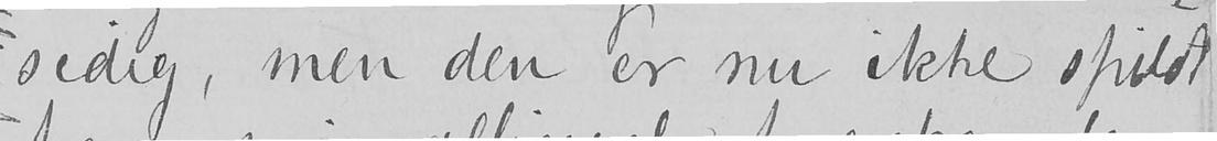sidig, men den er nu ikke spildt
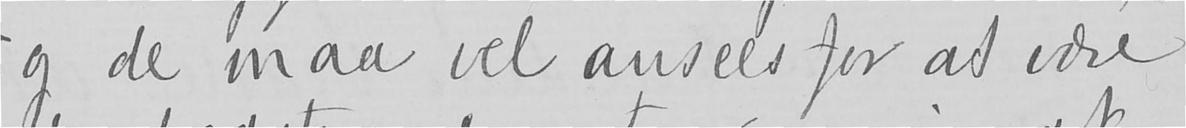og de maa vel ansees for at være
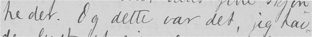heder. Og dette var det, jeg hav-
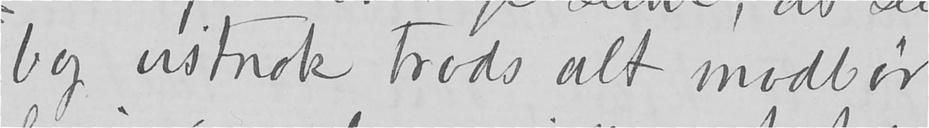bog vistnok trods alt modbør
